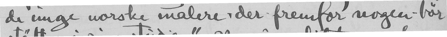de unge norske malere, der fremfor nogen - bør
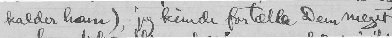kalder ham), - jeg kunde fortælla Dem meget
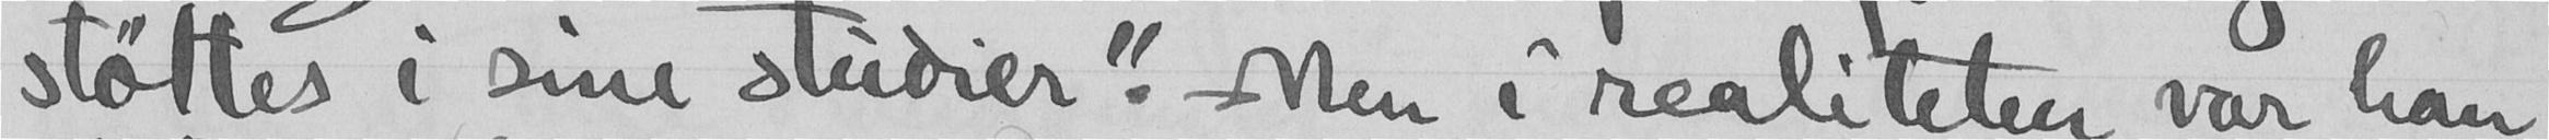støttes i sine studier". Men i realiteten var han
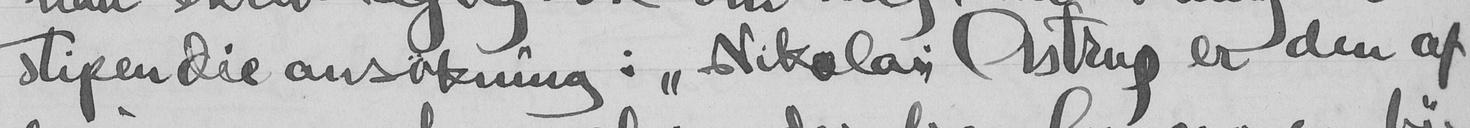stipendie ansøkning :" Nikolai Astrup er den af
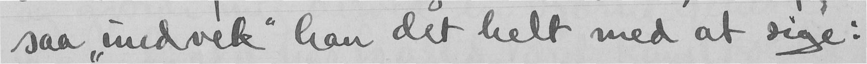saa "undvek" han det helt med at sige:
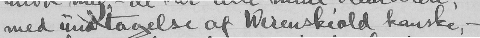med undtagelse af Werenskiold kanske, -
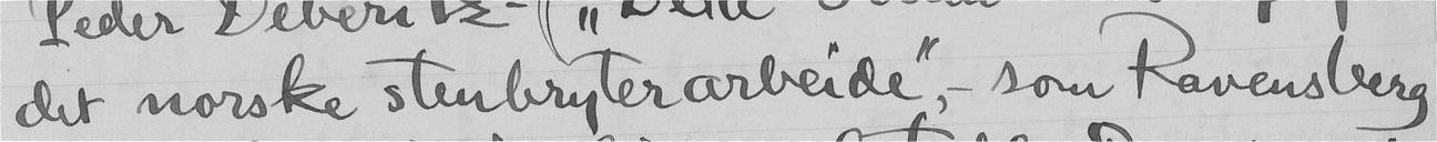det norske stenbryterarbeide", - som Ravensberg
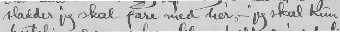sladder jeg skal fare med her, - jeg skal kun
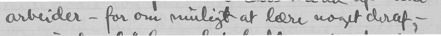arbeider - for om muligt at lære noget deraf, -
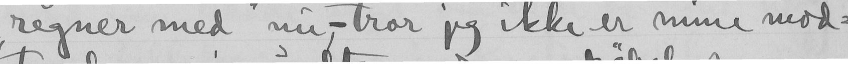"regner med" nu,- tror jeg ikke er mine mod-
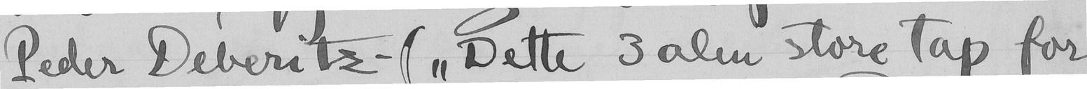Peder Deberitz - ("Dette 3 alm store tap for
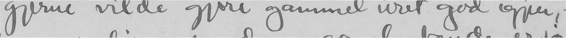gjerne vilde gjøre gammel uret god igjen,-
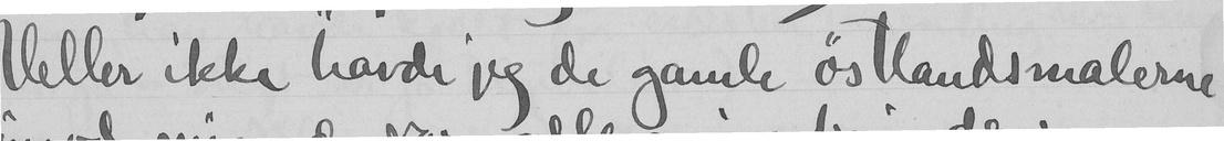Heller ikke havde jeg de gamle østlandsmalerne
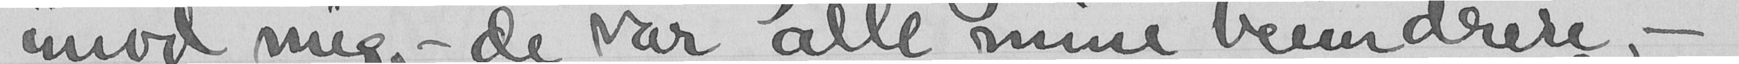imod mig - de var alle mine beundrere, -
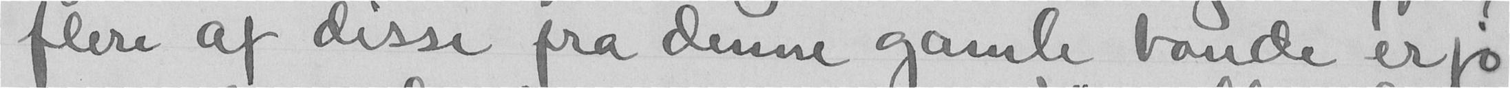flere af disse fra denne gamle bande er jo
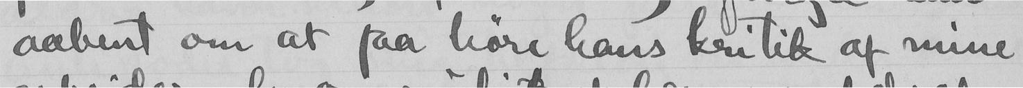aabent om at faa høre hans kritik af mine
